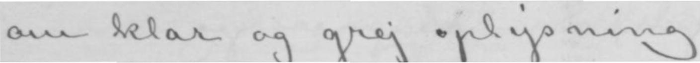om klar og grej oplysning.
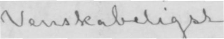Venskabeligst
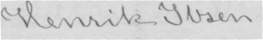Henrik Ibsen.
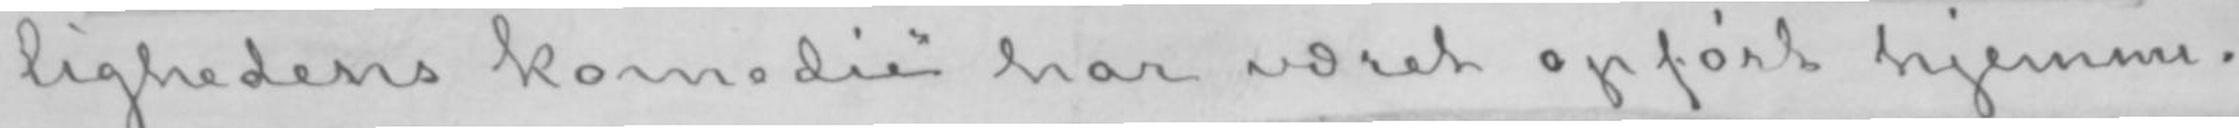lighedens komedie» har været opført hjemme.
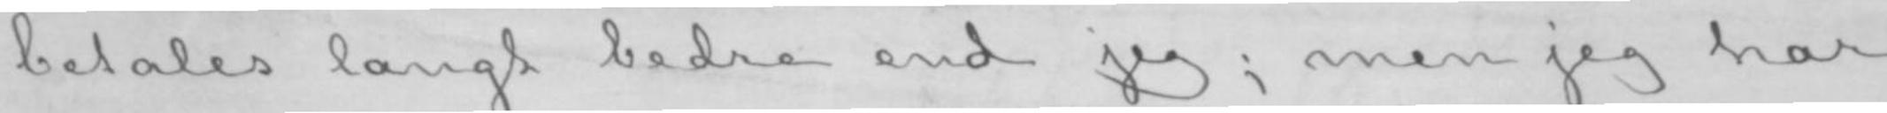betales langt bedre end jeg; men jeg har
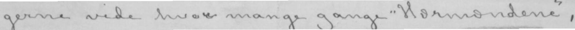gerne vide hvor mange gange «Hærmændene»,
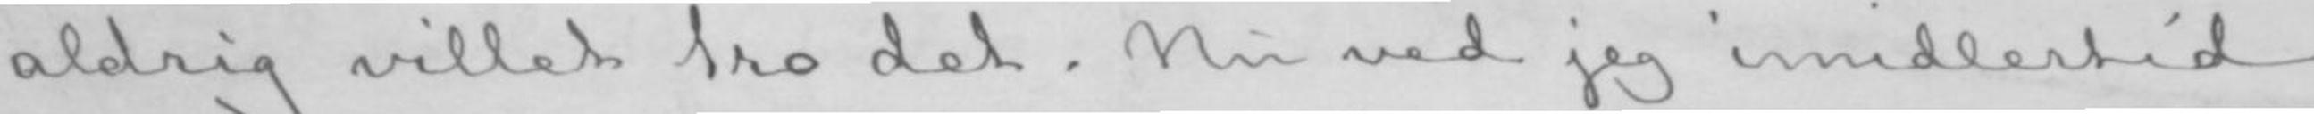aldrig villet tro det. Nu ved jeg imidlertid
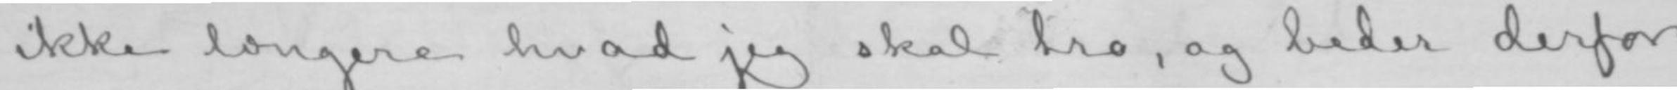ikke længere hvad jeg skal tro, og beder derfor
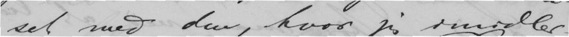set med den, hvor jeg imidler-
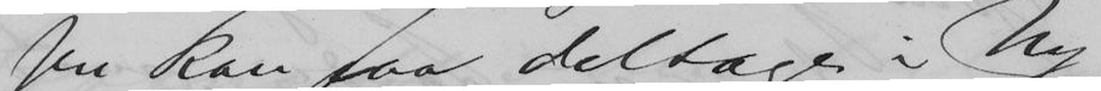sen kan faa deltage i Ny-
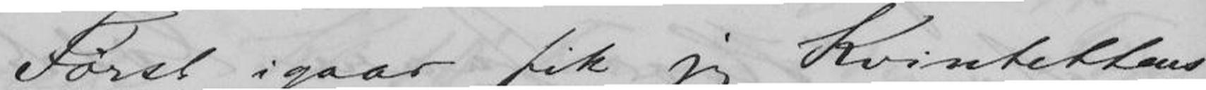Først igaar fik jeg Kvintettens
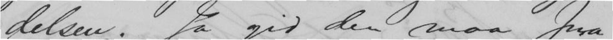delsen. Ja gid den maa sma-
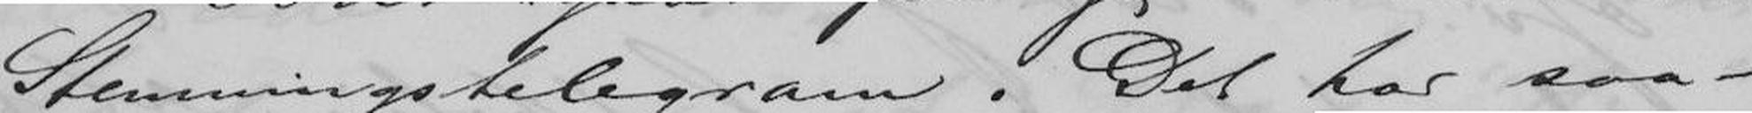Stemningstelegram. Det har saa-
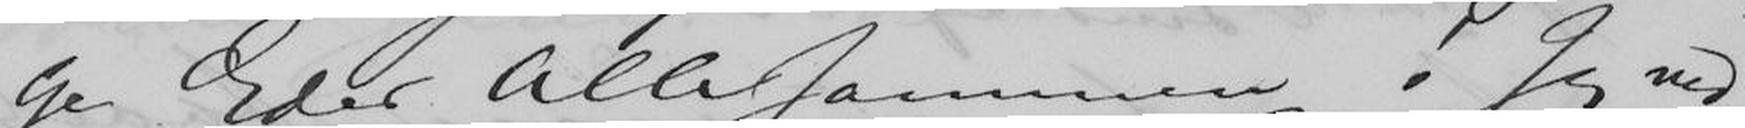ge Eder Allesammen. Jeg ved
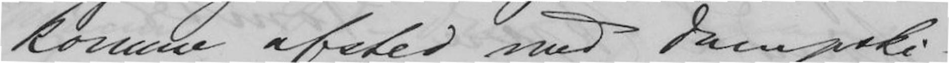komme afsted med Dampski-
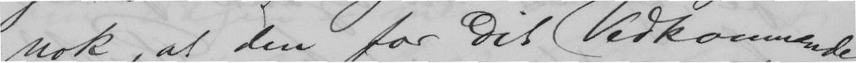nok, at den for Dit Vedkommende
